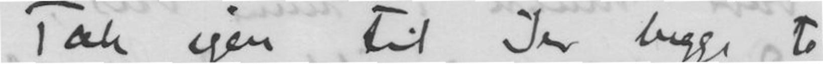Tak igen til Jer begge to
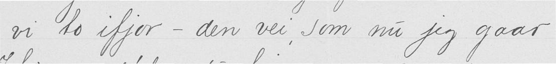- vi to ifjor - den vei, som nu jeg gaar - -
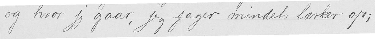og hvor jeg gaar, jeg jager mindets lærker op;
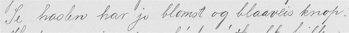Se haslen har jo blomst og blaaveis knop!
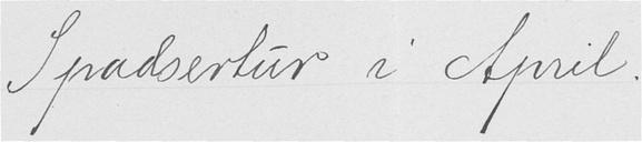Spadsertur i April.
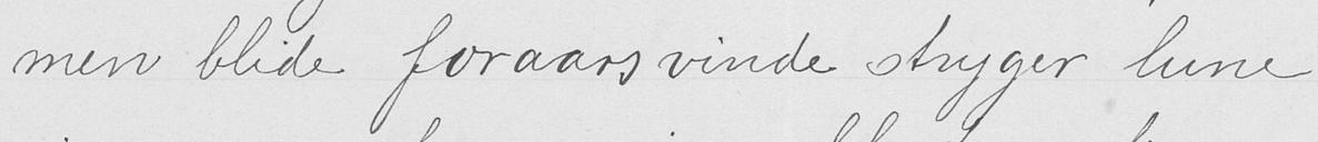men blide foraarsvinde stryger lune
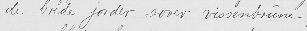de brede jorder sover vissenbrune;
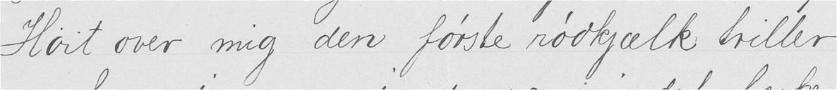Høit over mig den første rødkjælk triller
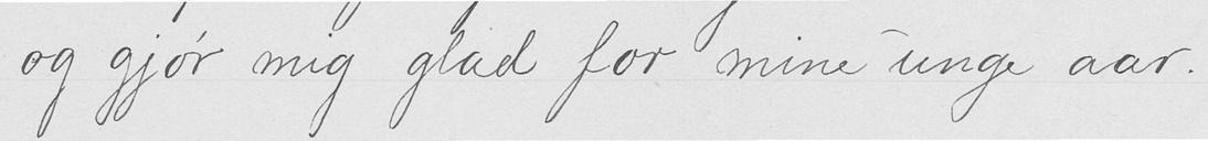og gjør mig glad for mine unge aar.
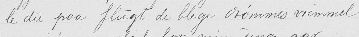le du paa flugt de blege drømmes vrimmel
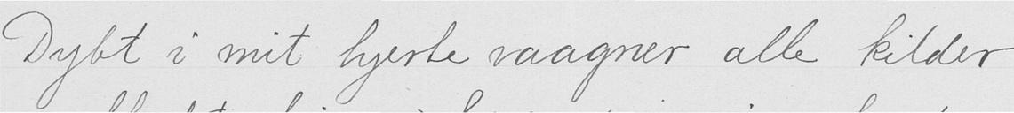Dybt i mit hjerte vaagner alle kilder
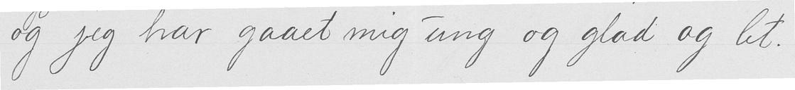og jeg har gaaet mig ung og glad og let.
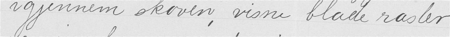igjennem skoven, visne blade rasler,
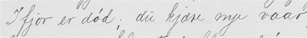Ifjor er død; du kjære nye vaar,
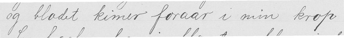og blodet kimer foraar i min krop -
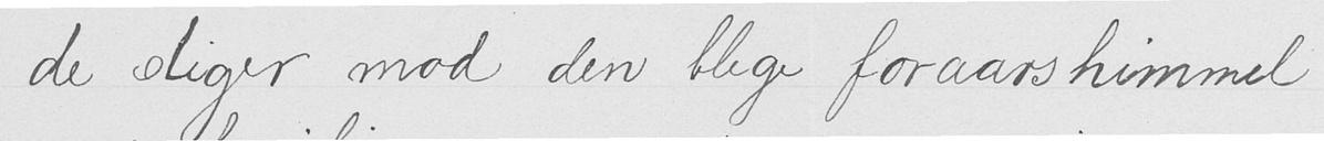de stiger mod den blege foraarshimmel
